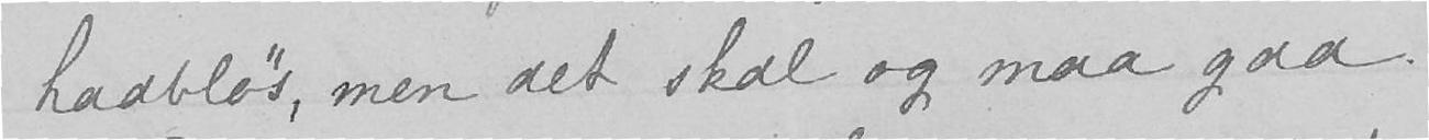haabløs, men det skal og maa gaa.
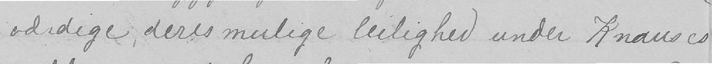værdige, deres mulige leilighed under Knauses
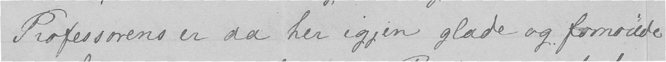Professorens er da her igjen glade og fornøiede
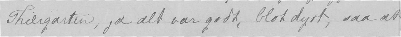Thiergarten, ja alt var godt, blot dyrt, saa at
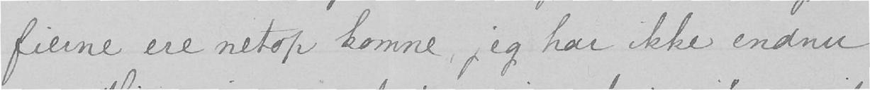fierne ere netop komne, jeg har ikke endnu
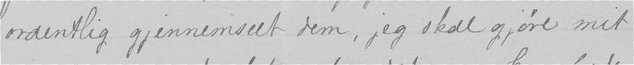ordentlig gjennemseet dem, jeg skal gjøre mit
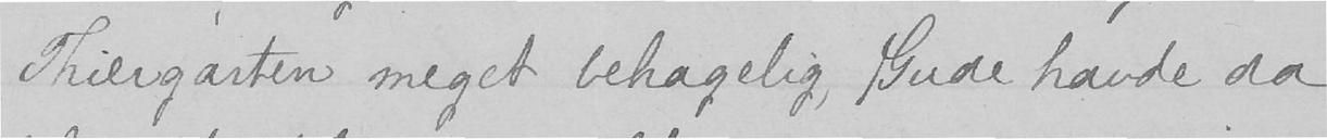Thiergarten meget behagelig, Gude havde da
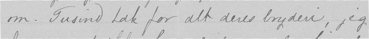om. Tusind tak for alt deres bryderi, jeg
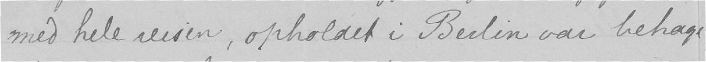med hele reisen, opholdet i Berlin var behage
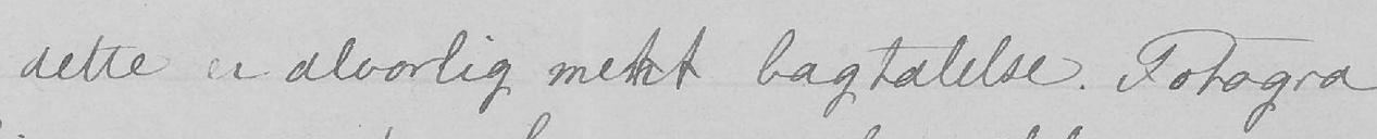dette er alvorlig ment bagtalelse. Fotogra-
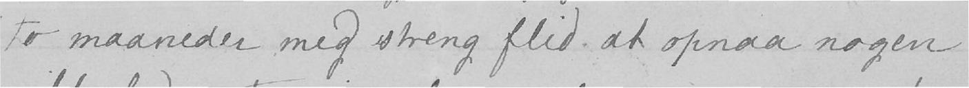to maaneder med streng flid at opnaa nogen
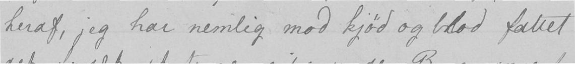heraf, jeg har nemlig mod kjød og blod fattet
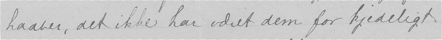haaber, det ikke har været dem for kjedeligt.
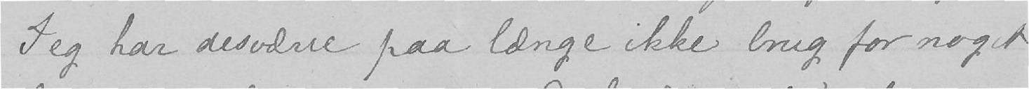Jeg har desværre paa længe ikke brug for noget
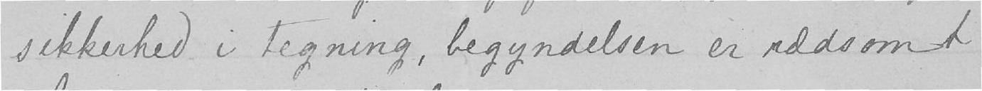sikkerhed i tegning, begyndelsen er rædsomt
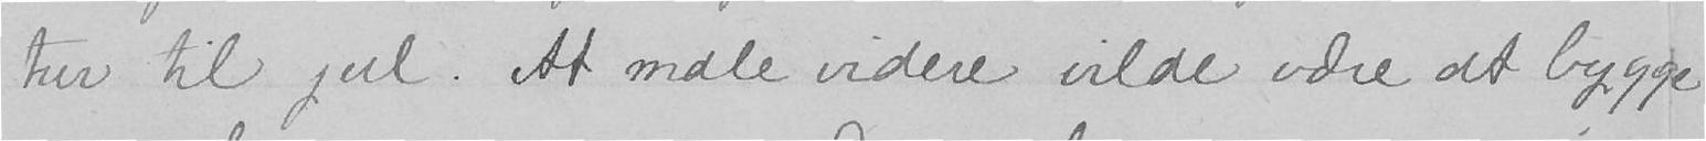tur til jul. At male videre vilde være at bygge
